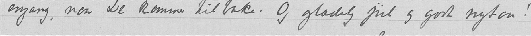engang, naar De kommer tilbake. Og glædelig jul og godt nytaar!
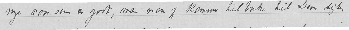nye iaar som er godt, men naar jeg kommer tilbake til Deres digte,
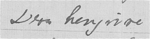Deres hengivne
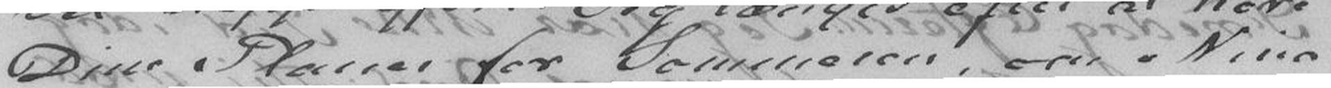Dine Planer for Sommeren, om Nina
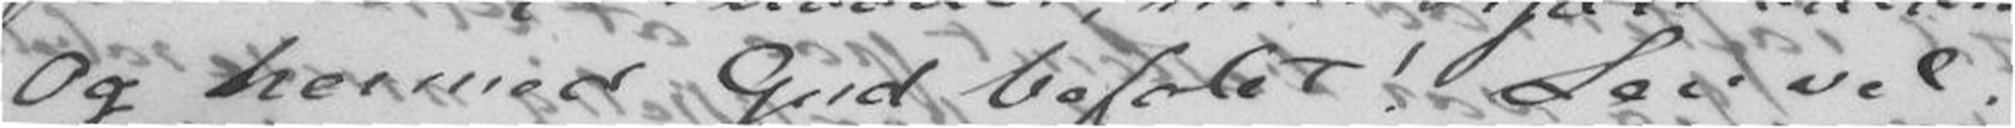Og hermed Gud befalet! Lev vel
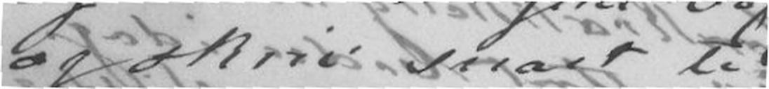og skriv snart til
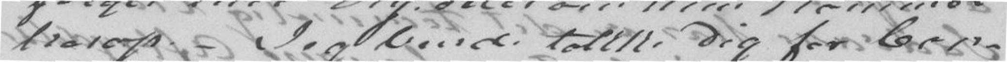herop - Jeg burde takke dig for Con-
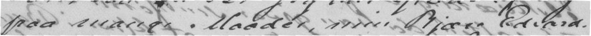paa mange Maader, min kjære Edvard.
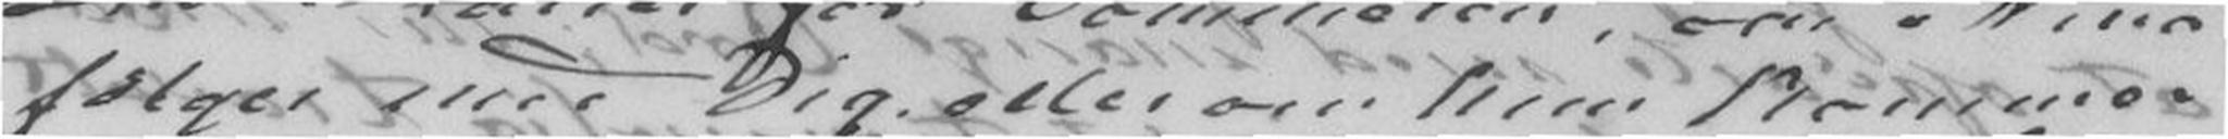følger med Dig eller om hun kommer
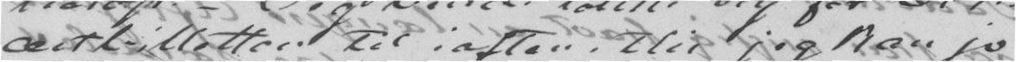certbilletter til iaften, thi jeg kan jo
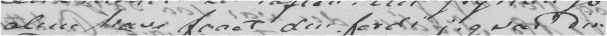alene have faaet den fordi jeg var Din
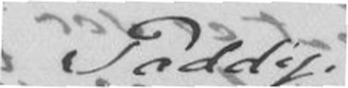Paddy.
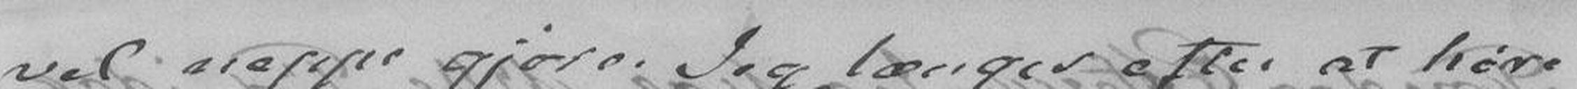vel neppe gjære. Jeg længes efter at høre
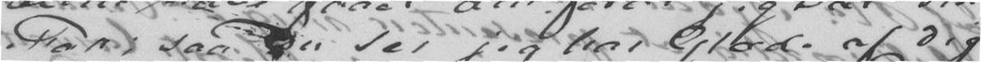Far, saa Du ser jeg har Glæde af Dig
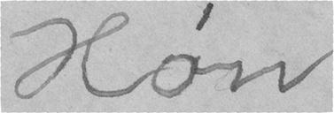Høn
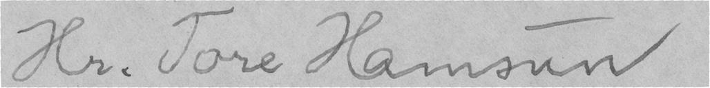Hr. Tore Hamsun
